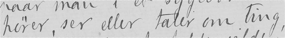hører, ser eller taler om ting,
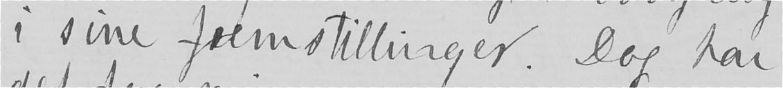i sine fremstillinger. Dog har
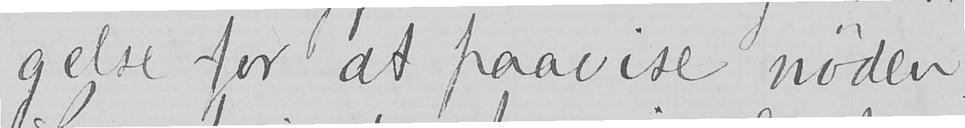gelse for at paavise nøden.
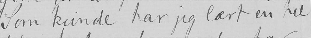Som kvinde har jeg lært en hel
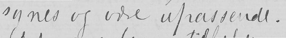synes og være upassende.
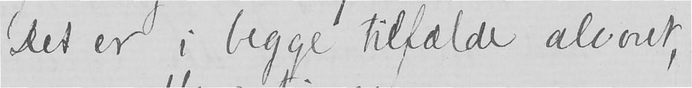Det er i begge tilfælde alvoret,
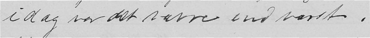i dag var det værre end værst.
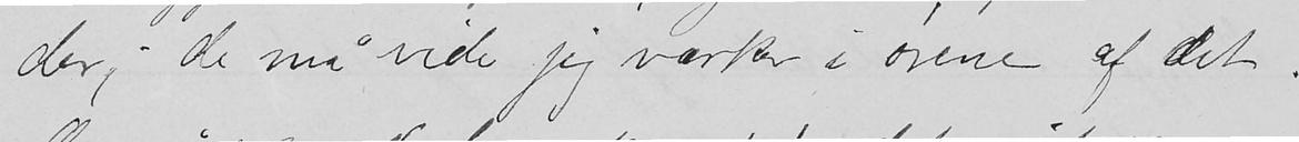der; de må vide jeg værker i ørene af det.
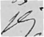jeg
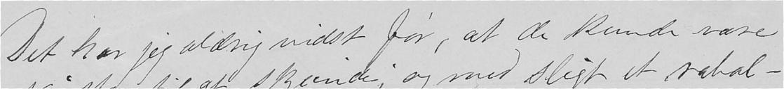Det har jeg aldrig vidst før, at de kunde være
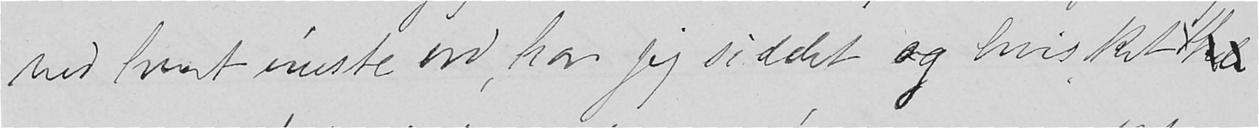ved hvert éneste ord, har jeg siddet og hvisket hele
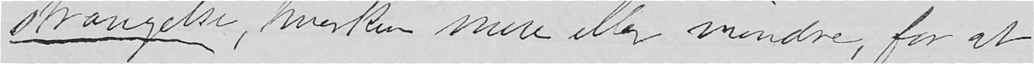strængelse, hverken mere eller mindre, for at
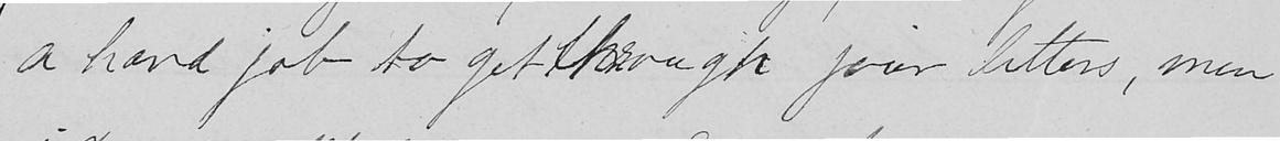a hard job to get through your letters, men
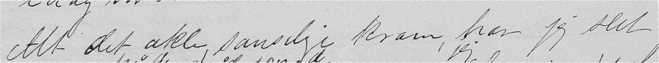Alt det ækle sanselige kram, har jeg slet
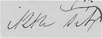ikke sét
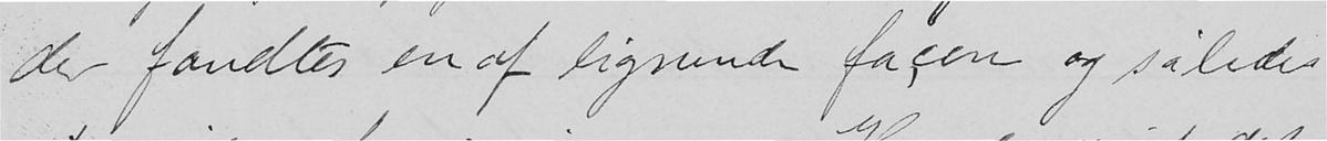der fandtes en af lignende façon og således
